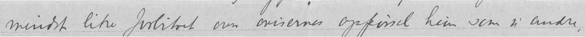mindst like forbitret over avisernes opførsel hun som vi andre,
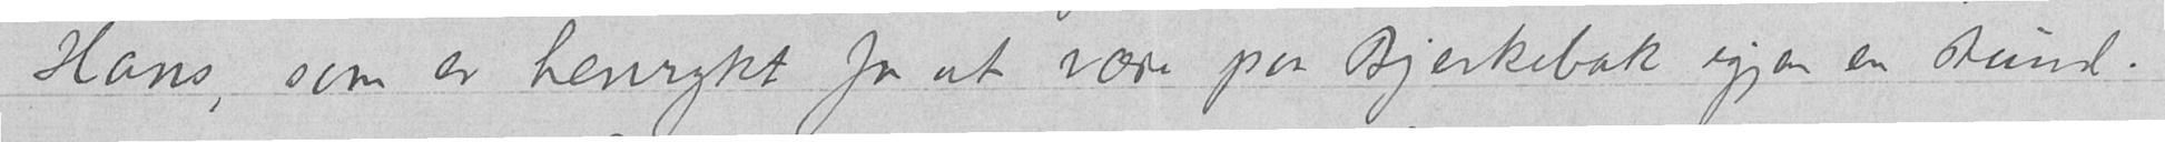Hans, som er henrykt for at være paa Bjerkebæk igjen en stund.
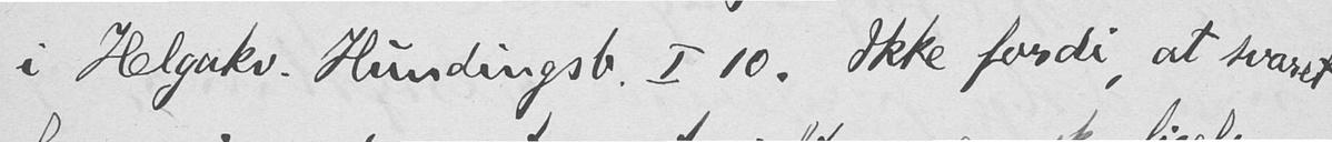i Helgakv. Hundingsb. I 10. Ikke fordi, at svaret
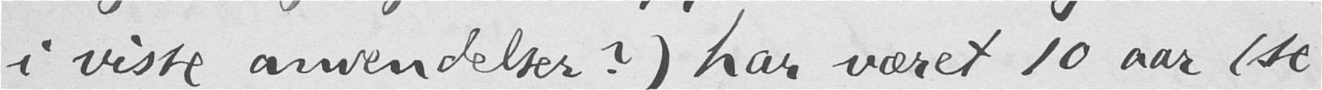i visse anvendelser?) har været 10 aar (se
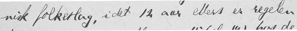nisk folkeslag, i det 12 aar ellers er regelen
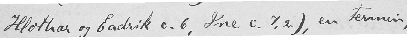Hlothar og Eadrik c. 6. Ine c. 7, 2), en termin,
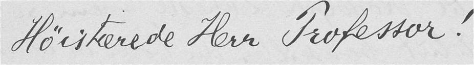Høistærede Herr Professor!
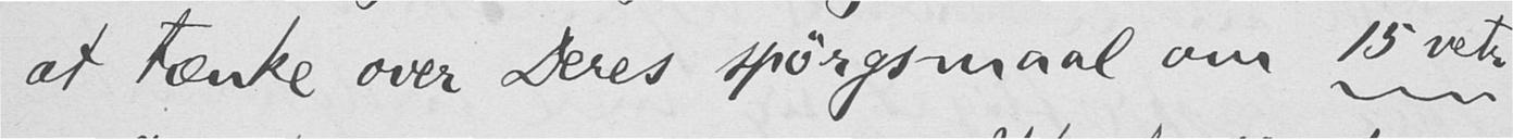at tænke over Deres spørgsmaal om 15 vetr
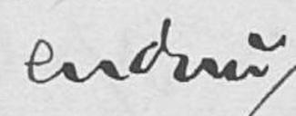endnu
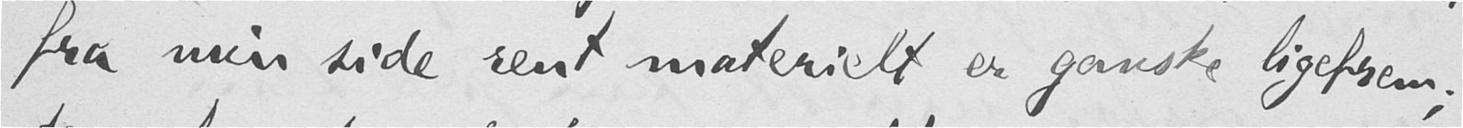fra min side rent materielt er ganske ligefrem;
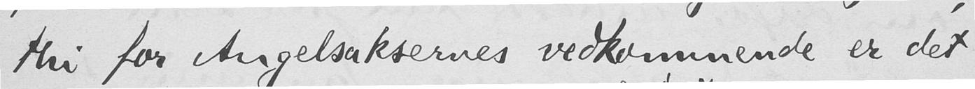thi for Angelsaksernes vedkommende er det
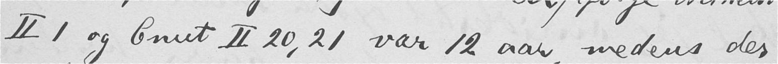II 1 og Cnut II 20, 21 var 12 aar, medens der
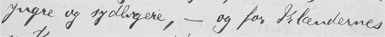yngre og sydligere, - og for Irlændernes
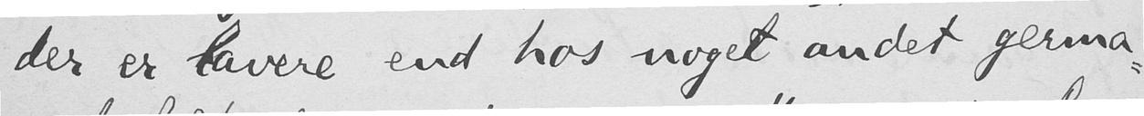der er lavere end hos noget andet germa-
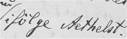ifølge Aethelst.
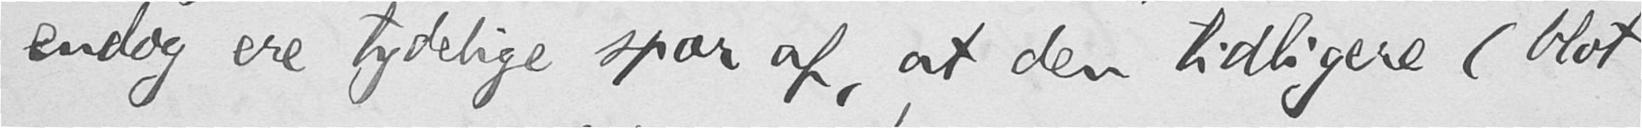endog ere tydelige spor af, at den tidligere (blot
